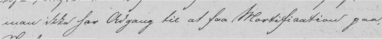man ikke har Adgang til at faa Mortification paa
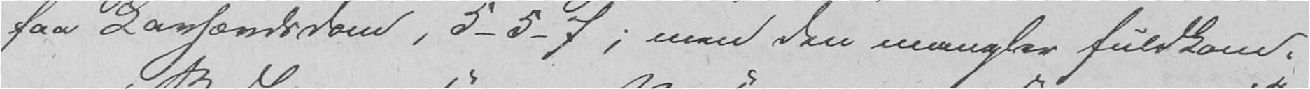faa Lovhævdsdom, 5-5-7; men den mangler fuldkom-
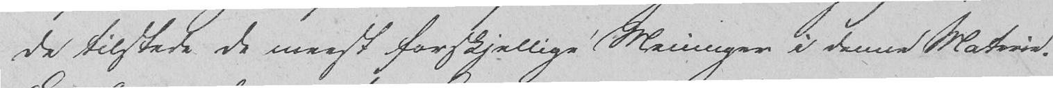de tilstede de meest forskjellige Meninger i denne Materie.
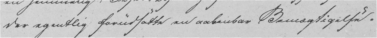der egentlig forudsatte en aabenbar Bemægtigelse.
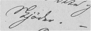Skjøder. -
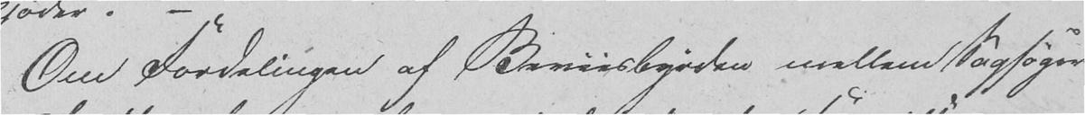Om Fordelingen af Beviisbyrden mellem Sagsøger
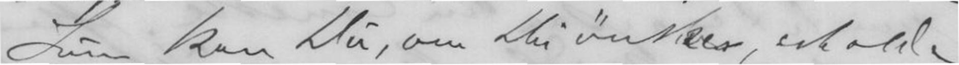Sum kan Du, om Du ønsker, erholde
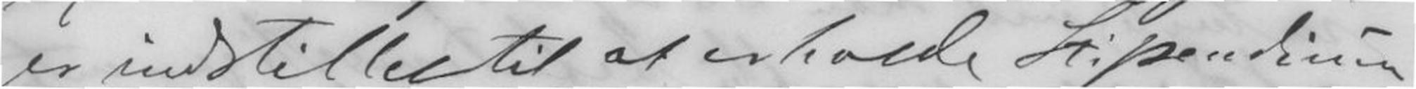er indstillet til at erholde Stipendium
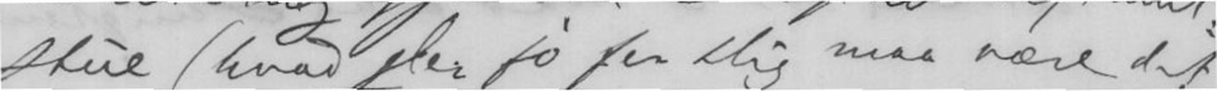stue (hvad der jo for Dig maa være det
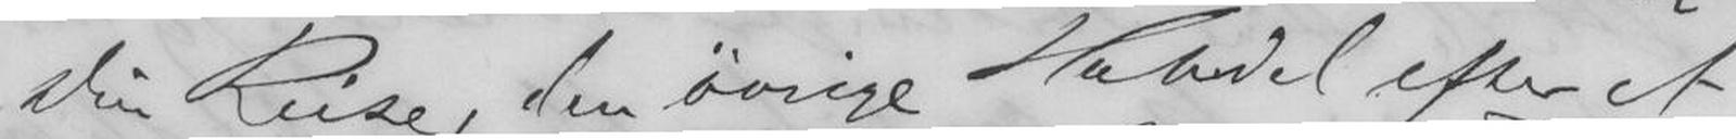Din Reise, den øvrige Halvdel efter et
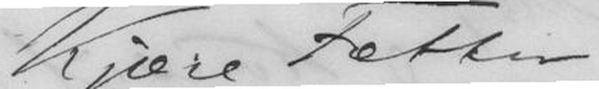Kjære Fætter
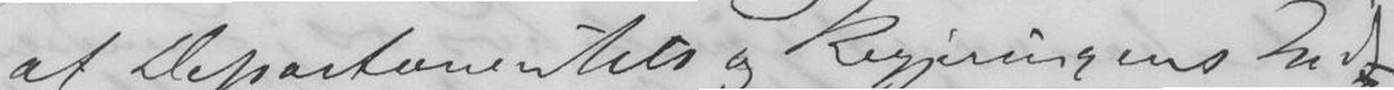at Departementets og Regjeringens Ind-
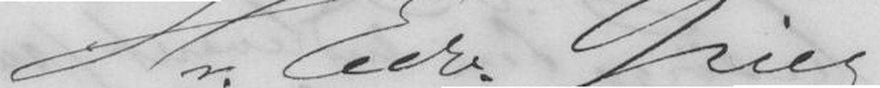Hr. Edvard Grieg
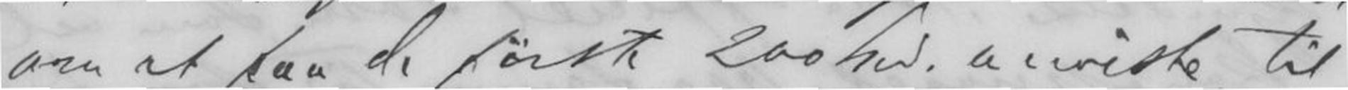om at faa de første 200Spd. anviste til
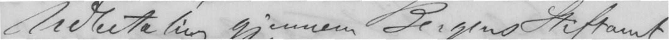Udbetaling gjennem Bergens Stiftamt-
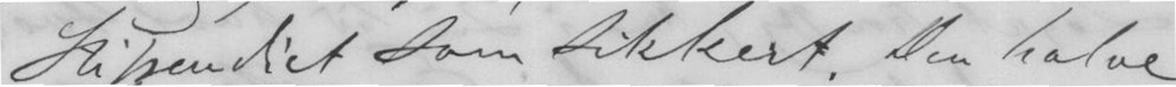Stipendiet som sikkert. Den halve
